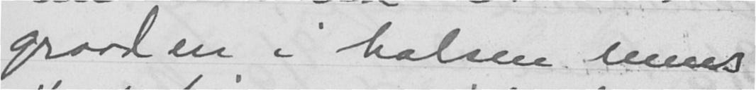graaden i halsen mens
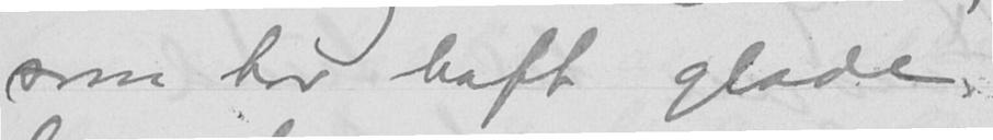som har haft glade
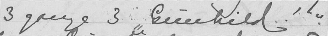3 gange 3 "Gunhild!"
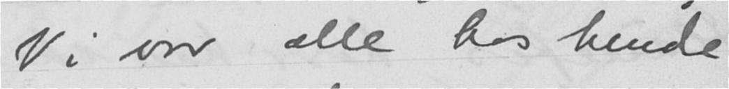Vi var alle hos hende
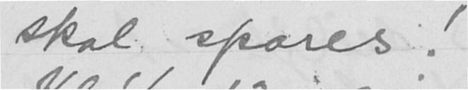skal spares!
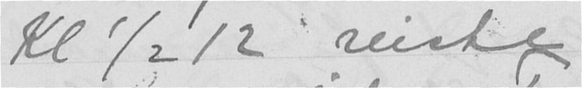Kl ½ 12 reiste
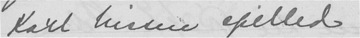Karl Nissen spilled
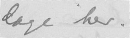dage her.
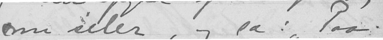som siler, og sa: "Paa
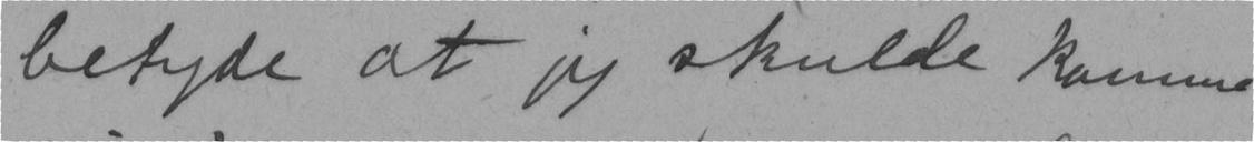betyde at jeg skulde komme
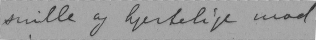snille og hjertelige mod
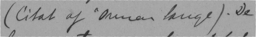(Citat af "Ormen lange). De
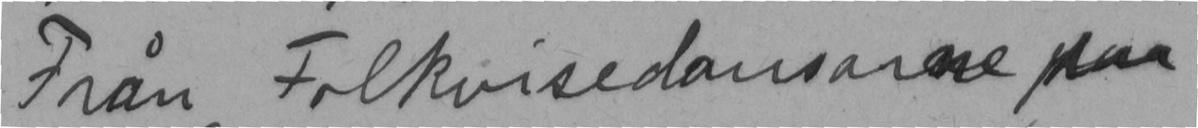Från Folkvisedansarne paa
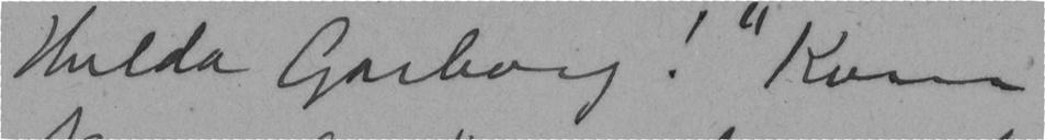Hulda Garborg! "Kom
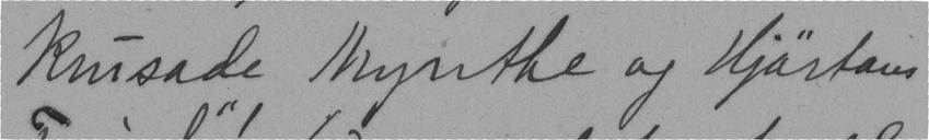Krusade Mynthe og Hjørtans
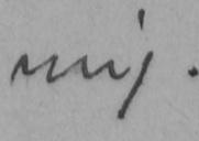mig.
